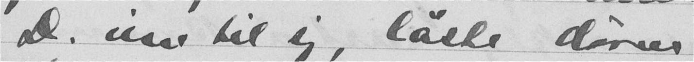D. inn til sig, låste døren
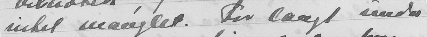intet manglet. For langt under
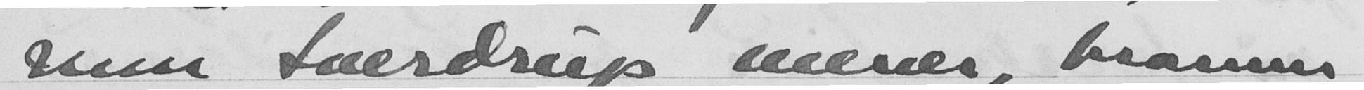men Sverdrup mener, brannen
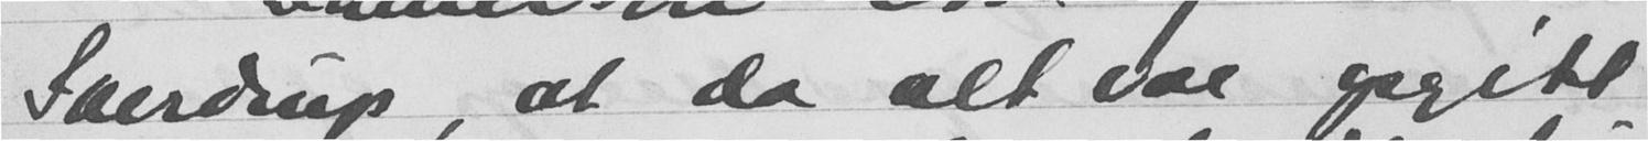Sverdrup, at da alt var opgitt
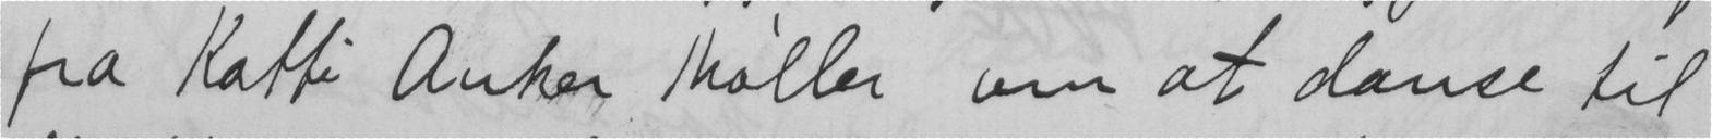fra Katti Anker Møller om at danse til
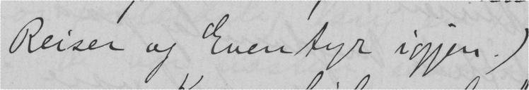Reiser og Eventyr igjen.)
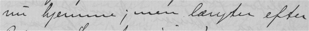nu hjemme; men længter efter
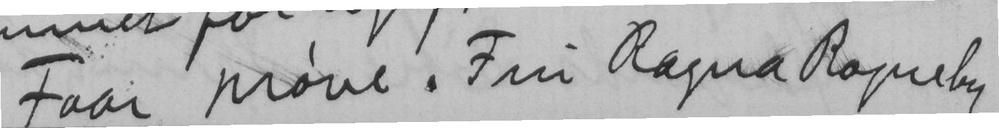Faar prøve. Fru Ragna Rogneby
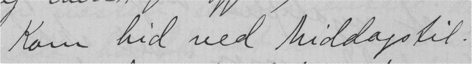Kom hid ved Middagstid.
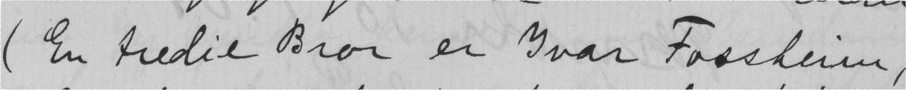En tredie Bror er Ivar Fossheim,
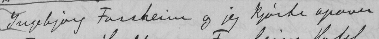Ingebjorg Forssheim og jeg kjørte opover
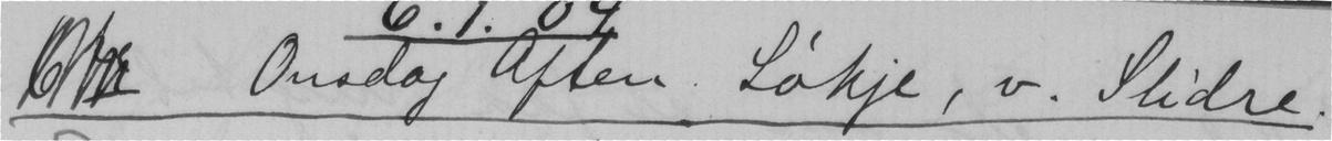Onsdag Aften. Løkje, v. Slidre.
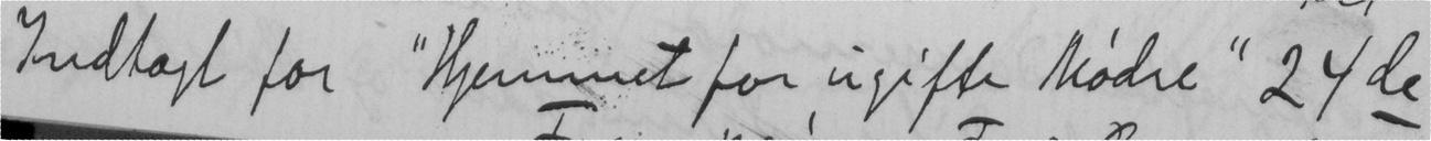Indtægt for "Hjemmet for ugifte Mødre" 24de
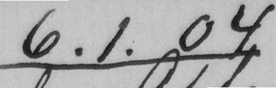6.1.04
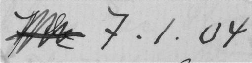7.1.04
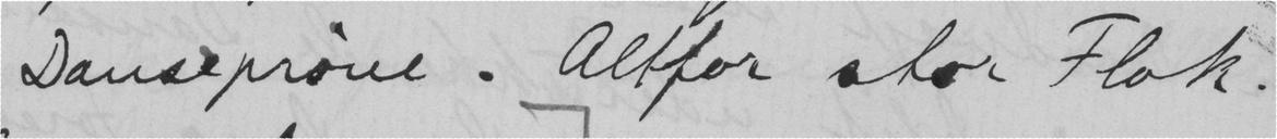Danseprøve. Altfor stor Flok.
